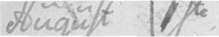August 1ste
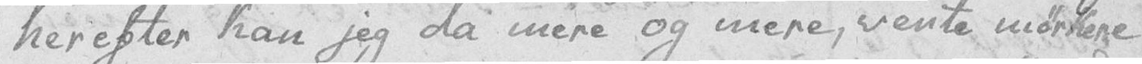herefter kan jeg da mere og mere, vente mørkere
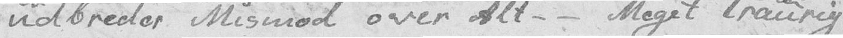udbreder Mismod over Alt. – Meget traurig
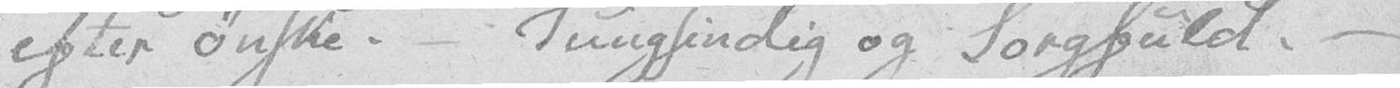efter ønske. – Tungsindig og Sorgfuld. –
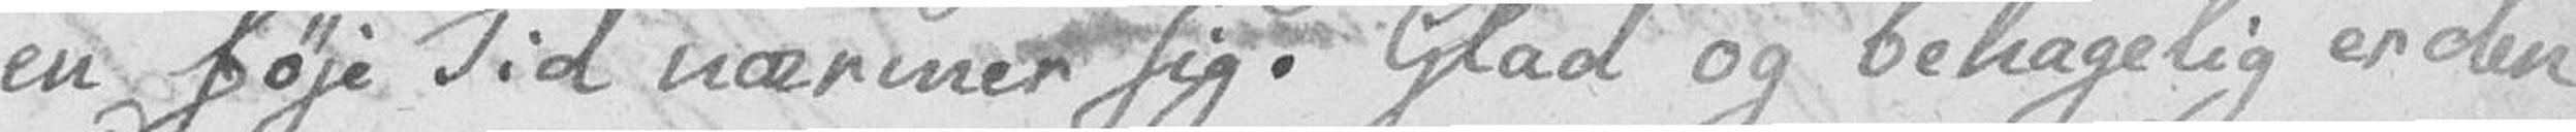en føje Tid nærmer sig. Glad og behagelig er den
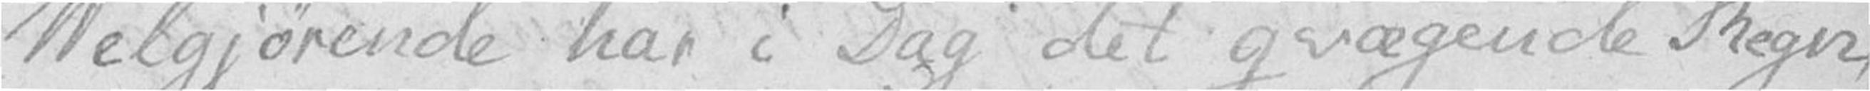Velgjørende har i Dag det qvægende Regn,
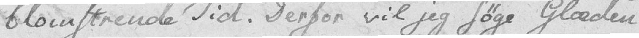blomstrende Tid. Derfor vil jeg søge Glæden
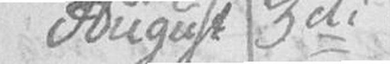August 3di
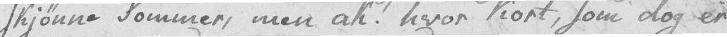skjønne Sommer, men ak! hvor kort, som dog er
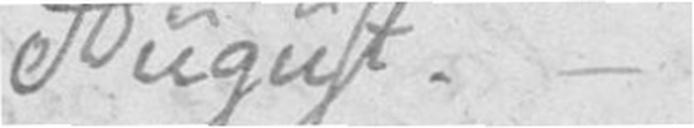August. –
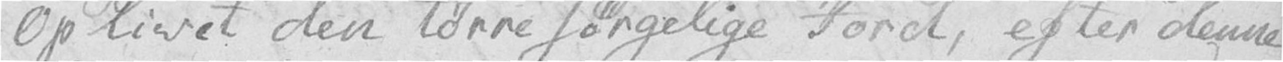oplivet den tørre sørgelige Jord, efter denne
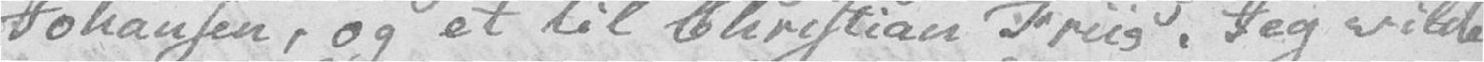Johansen, og et til Christian Friis. Jeg vilde
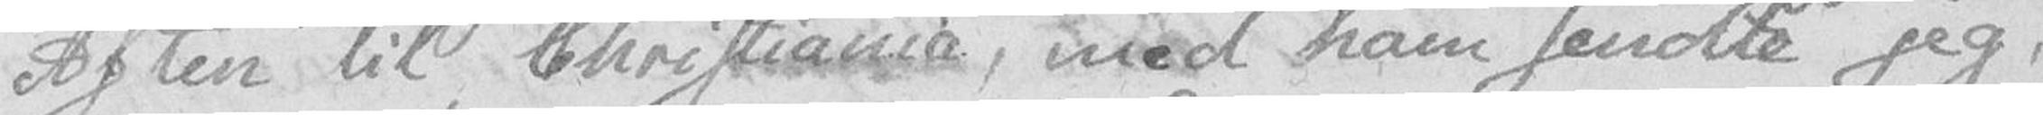Aften til Christiania, med ham sendte jeg,
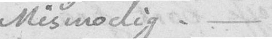Mismodig. –
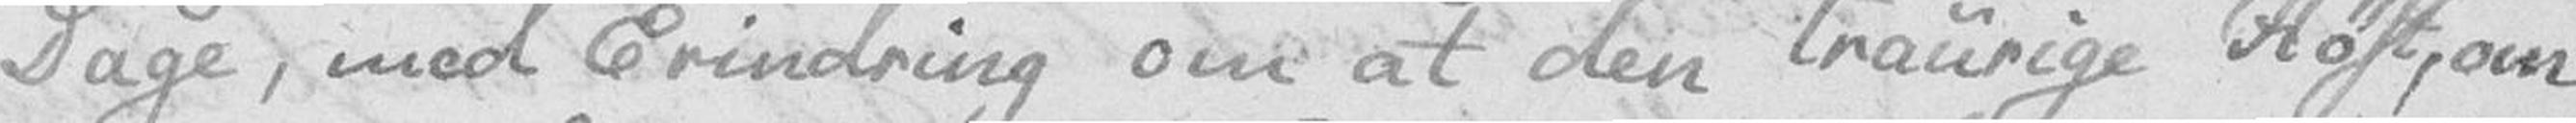Dage, med Erindring om at den traurige Høst, om
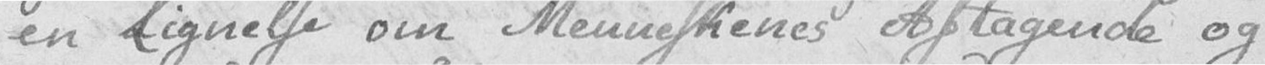en Lignelse om Menneskenes Aftagende og
